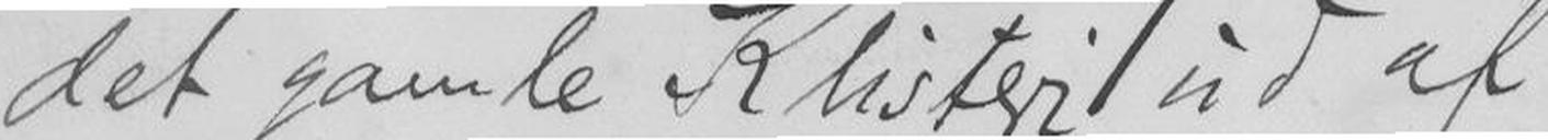det gamle Klisteri ud af
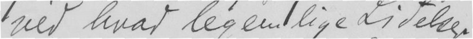ved hvad legemlige Lidelser
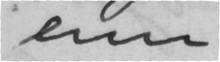enn
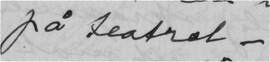på teatret —
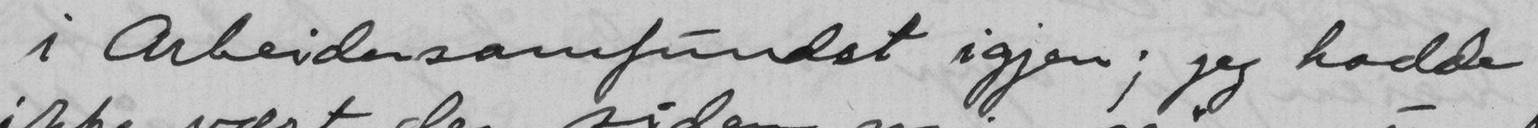i Arbeidersamfundet igjen; jeg hadde
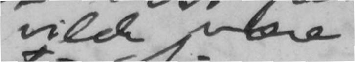vilde være
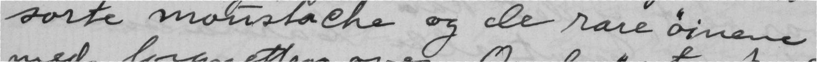sorte moustache og de rare øinene
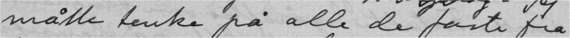måtte tenke på alle de faste fra
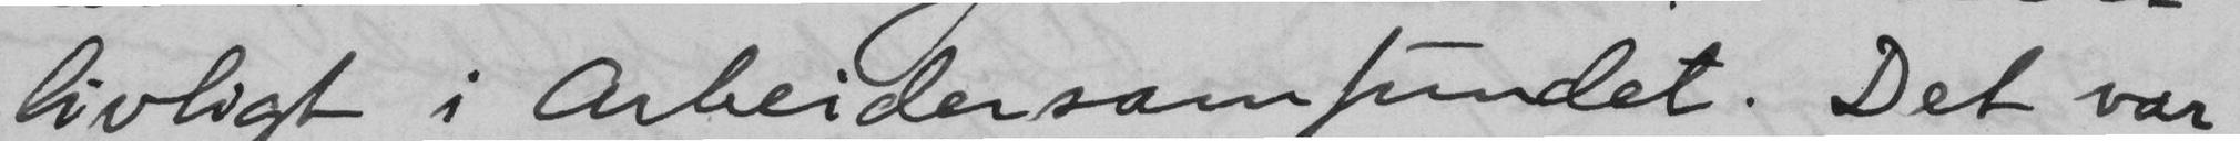livligt i Arbeidersamfundet. Det var
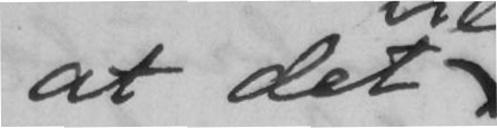at det
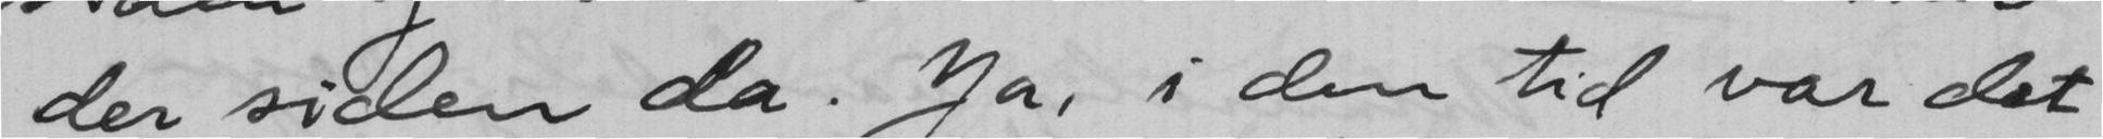der siden da. Ja, i den tid var det
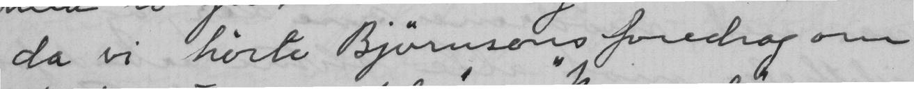da vi hørte Bjørnsons foredrag om
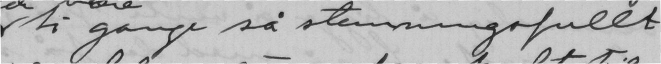ti gange så stemningsfullt
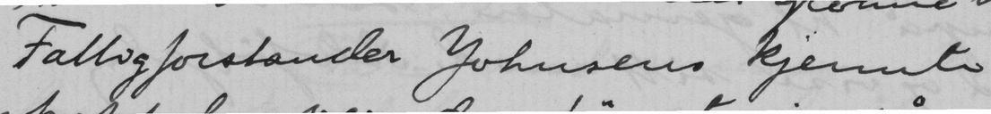Fattigforstander Johnsens kjente
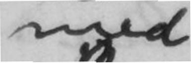med
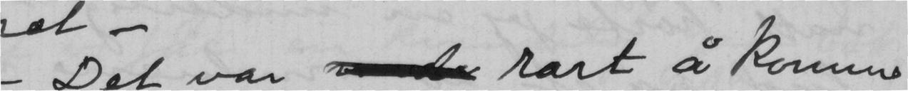- Det var  rart å komme
